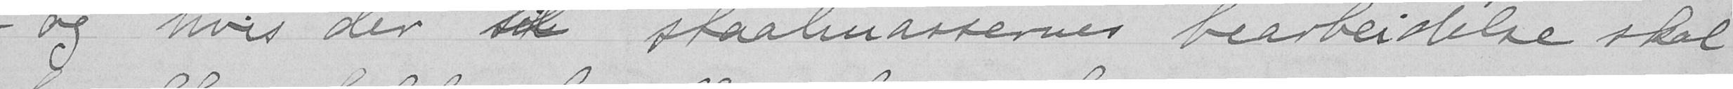og hvis der til staalmasternes bearbeidelse skal
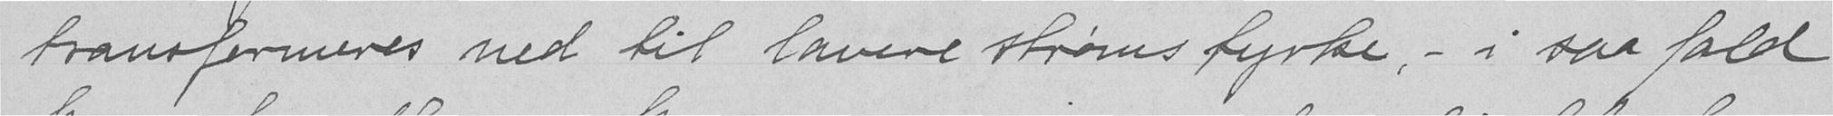transformeres ned til lavere strømstyrke, - i saa fald
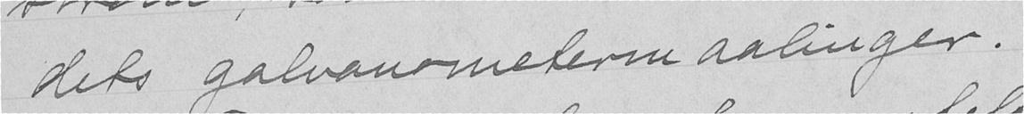dets galvanometernemaalinger.
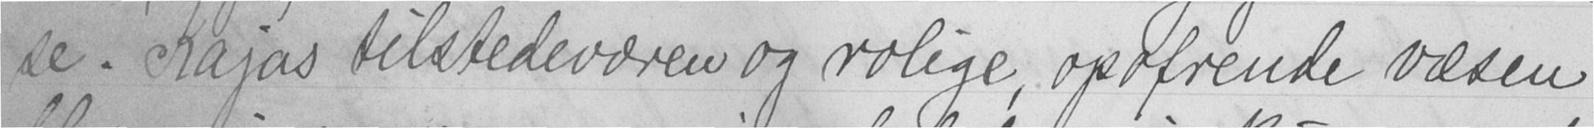se. Kajas tilstedeværen og rolige, opofrende væsen
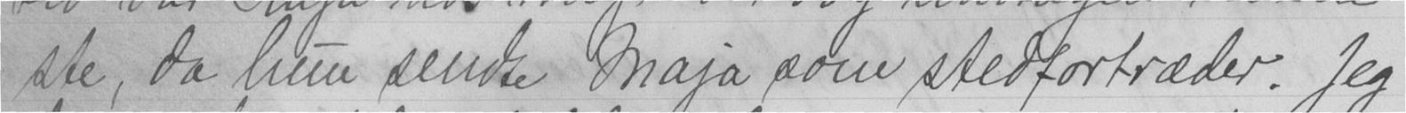ste, da hun sendte Maja som stedfortræder. Jeg
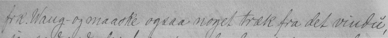frk. Wang og maaske ogsaa noget træk fra det vindu,
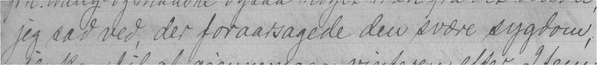jeg sad ved, der foraarsagede den svære sygdom,
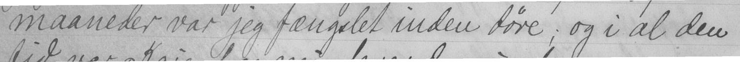maaneder var jeg fængslet inden døre; og i al den
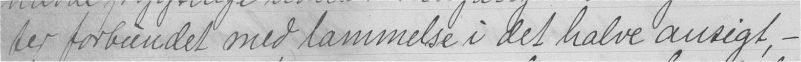ter forbundet med lammelse i det halve ansigt, -
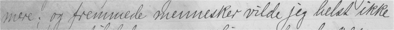mere; og fremmede mennesker vilde jeg helst ikke
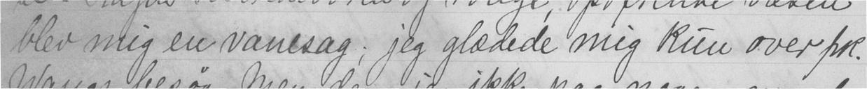blev mig en vanesag; jeg glædede mig kun over frk.
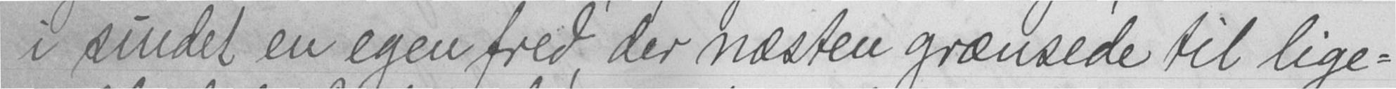i sindet en egen fred, der næsten grænsede til lige-
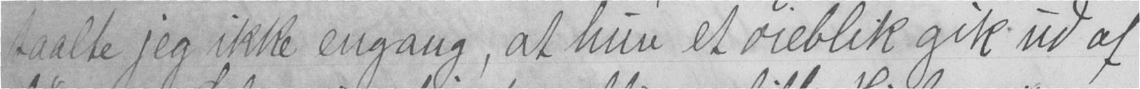taalte jeg ikke engang, at hun et øieblik gik ud af
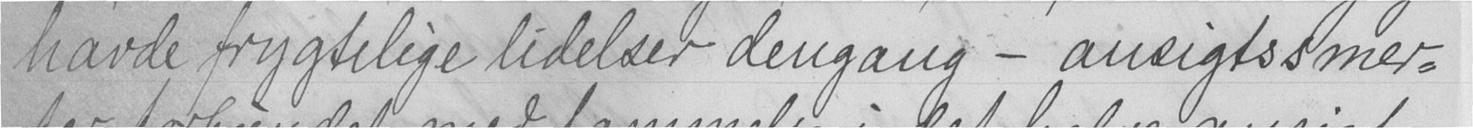havde frygtelige lidelser dengang - ansigtssmer-
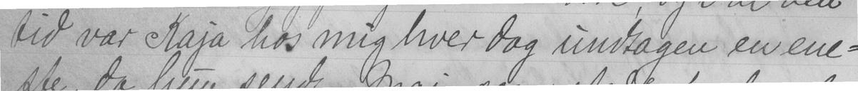tid var Kaja hos mig hver dag undtagen en ene-
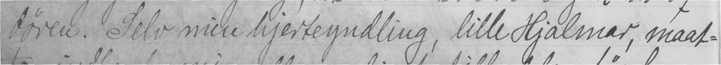døren. Selv min hjerteyndling, lille Hjalmar, maat-
